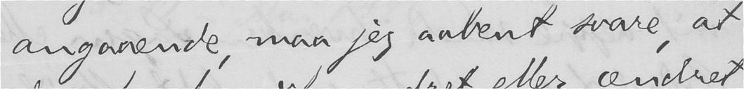angaaende, maa jeg aabent svare, at
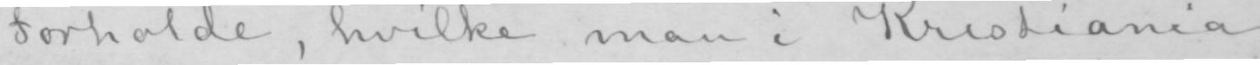Forholde, hvilke man i Kristiania
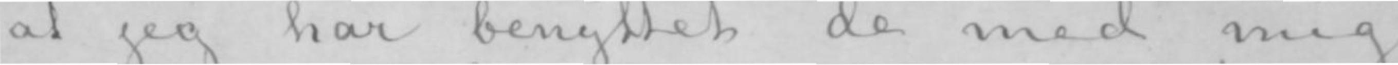at jeg har benyttet de med mig
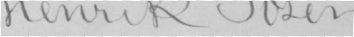Henrik Ibsen.
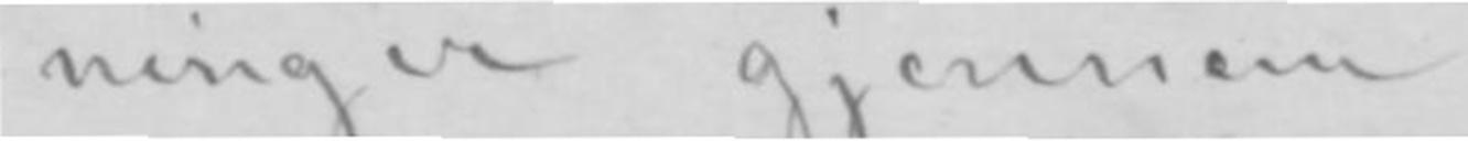ninger gjennem
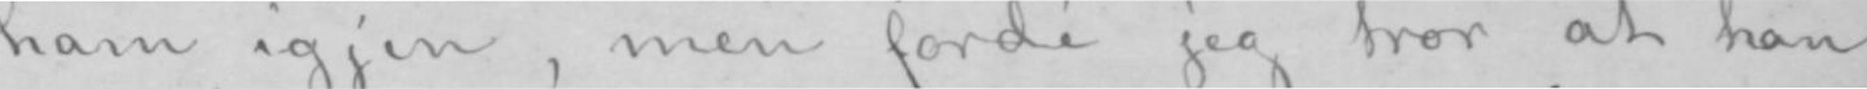ham igjen, men fordi jeg tror at han
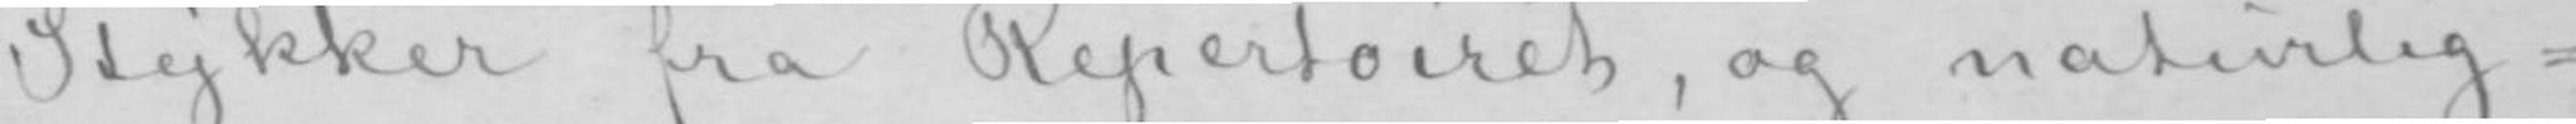Stykker fra Repertoiret, og naturlig-
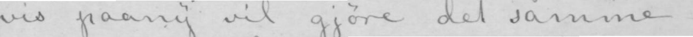vis paany vil gjøre det samme.
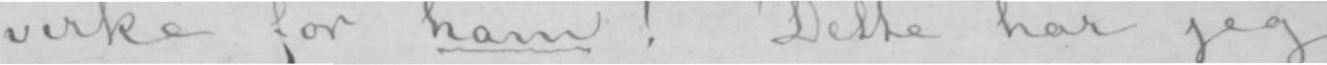virke for ham! Dette har jeg
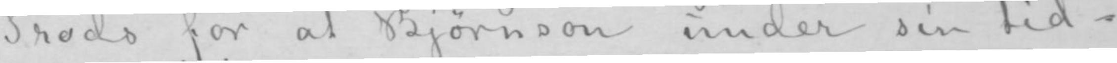Trods for at Bjørnson under sin tid-
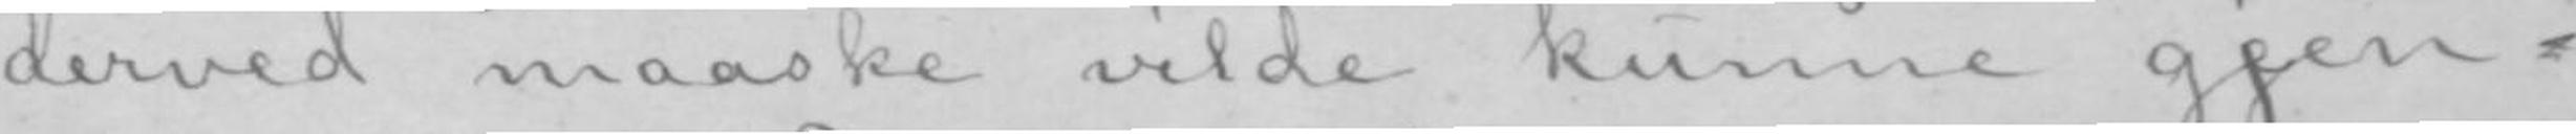derved maaske vilde kunne gjen-
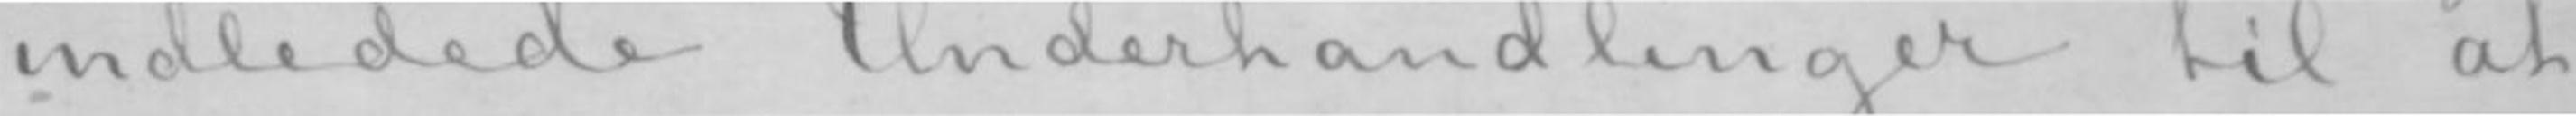indledede Underhandlinger til at
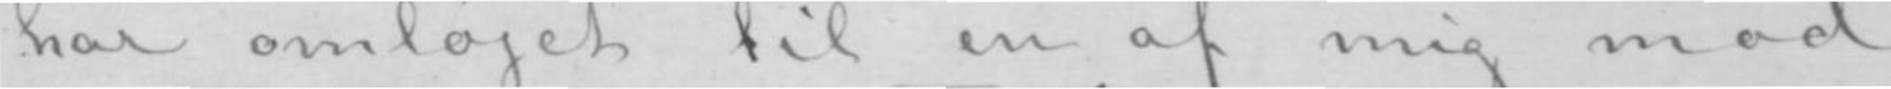har omløjet til en af mig mod
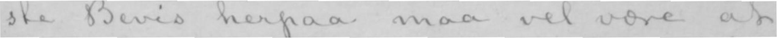ste Bevis herpaa maa vel være at
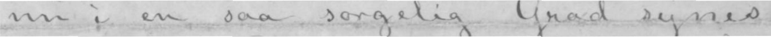nu i en saa sørgelig Grad synes
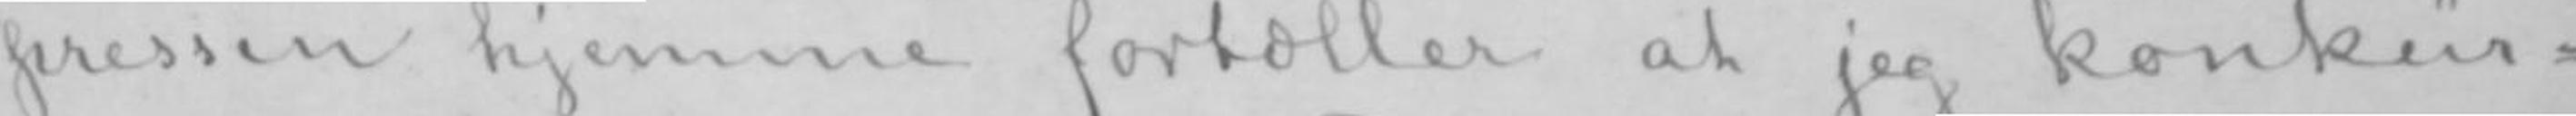pressen hjemme fortæller at jeg konkur-
